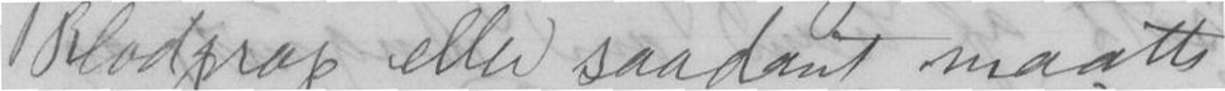Blodprop eller saadant maatte
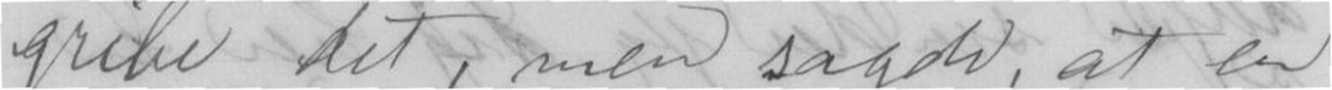gribe det, men sagde, at en
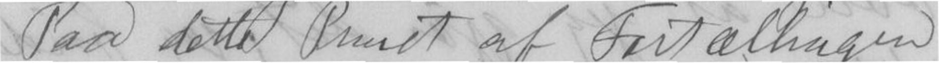Paa dette Punkt af Fortællingen
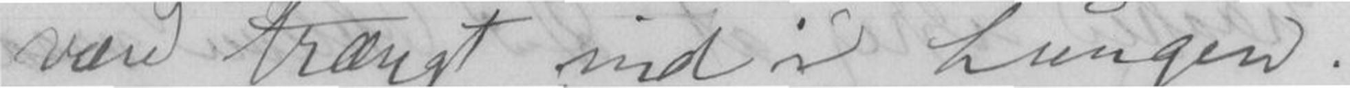være trængt ind i Lungen.
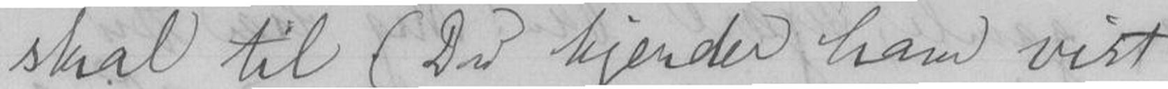skal til (Du kjender ham vist
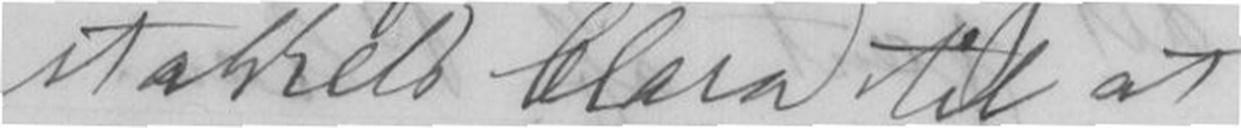stakkels Clara til at
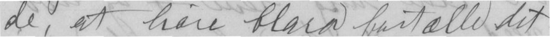de, at høre Clara fortælle det.
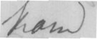kom
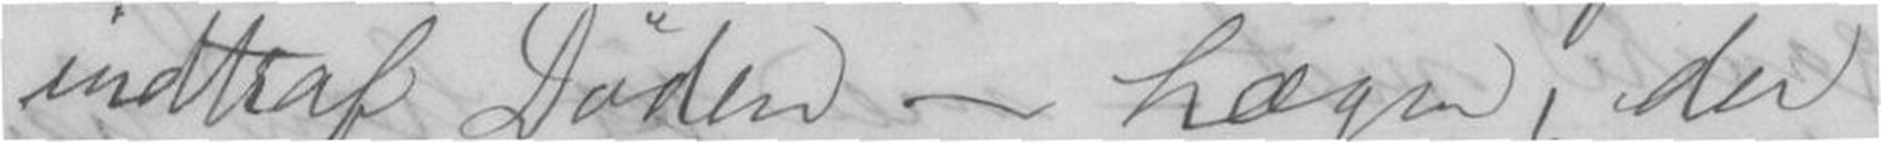intraf Døden - Lægen, der
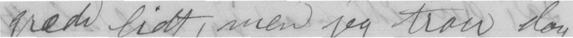græde lidt, men jeg tror dog
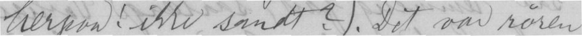herpaa ! ikke sandt ?). Det var røren
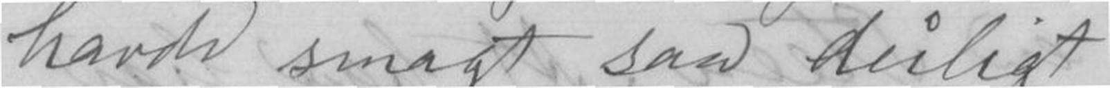havde smagt saa deiligt
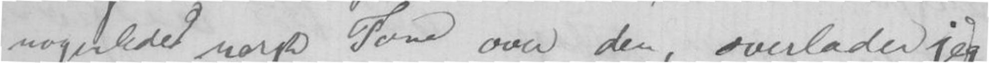nogenledes norsk Tone over den, overlader jeg
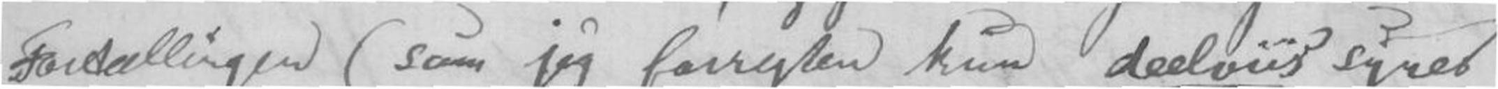Fortællingen (som jeg forresten kun deelviis synes
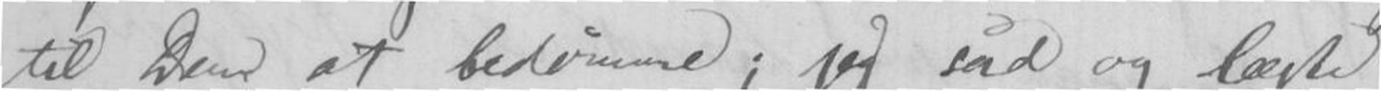til Dem at bedømme; jeg sad og læste
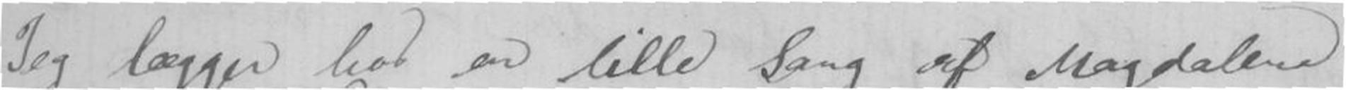Jeg lægger ved en lille Sang af Magdalene
